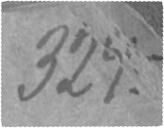329
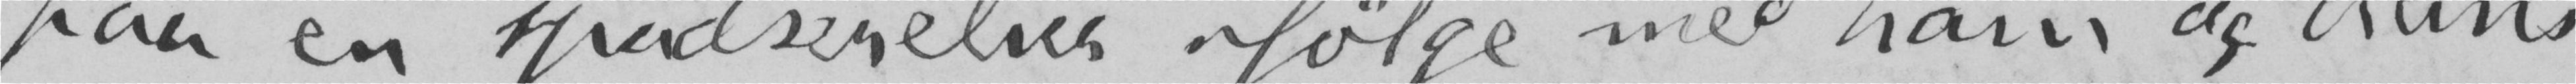paa en spadseretur ifølge med ham og hans
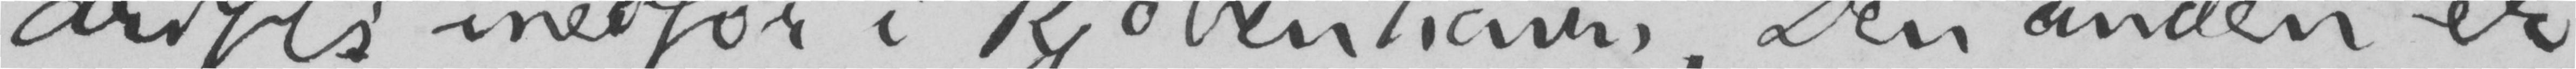drifts medfør i Kjøbenhavn. Den anden er
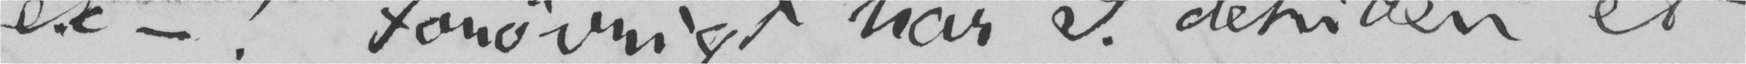ex –! Forøvrigt har S. desuden et
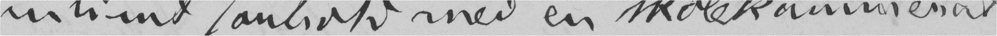intimt <sam…??> med en skolekammerat
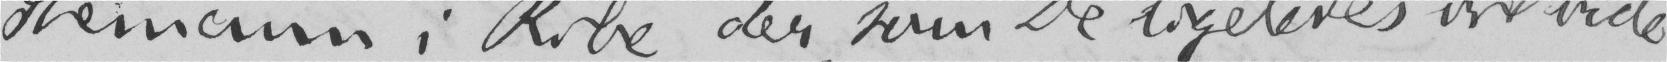Stemann i Ribe, der, som De ligeledes vil vide,
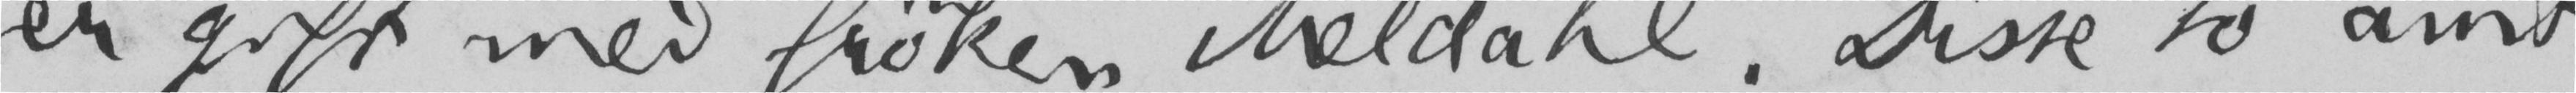er gift med frøken Meldahl. Disse to amt-
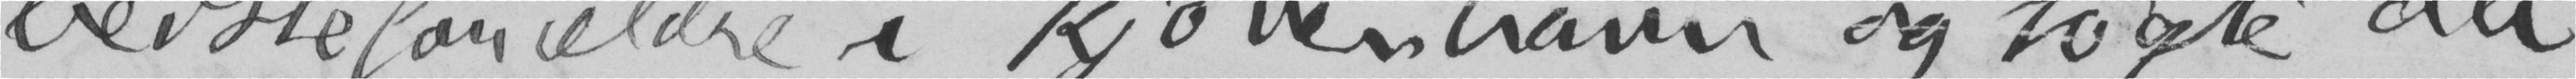bedsteforældre i Kjøbenhavn og søgte da
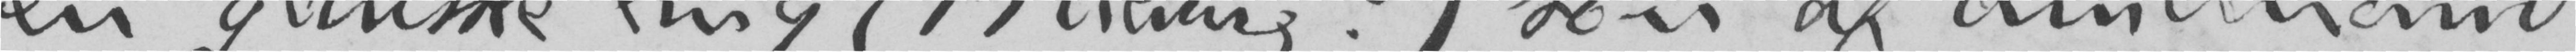en ganske ung (17 aarig?) søn af amtmand
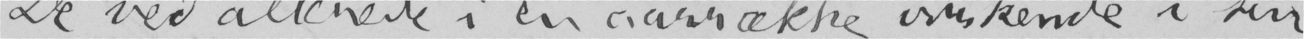De ved allerede i en aarrække virkende i sin
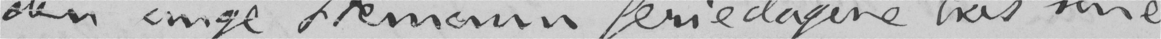den unge Stemann feriedagene hos sine
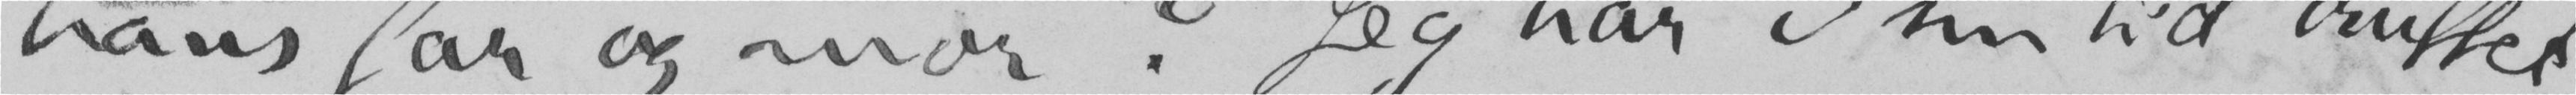hans far og mor? Jeg har i sin tid truffet
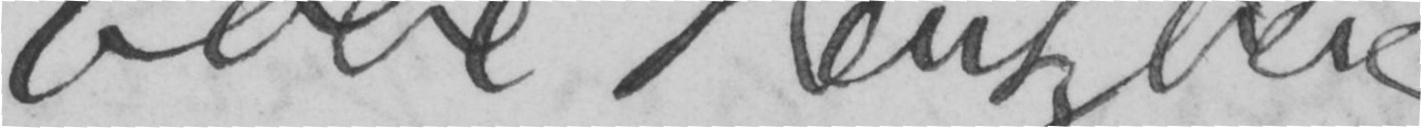Ebbe Hertzberg
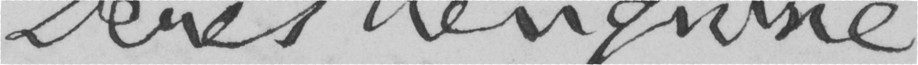Deres hengivne
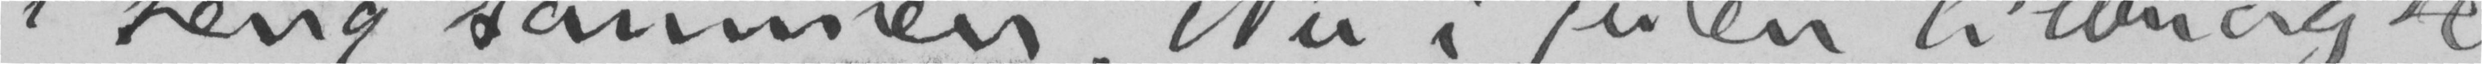i seng sammen. Nu i julen tilbragte
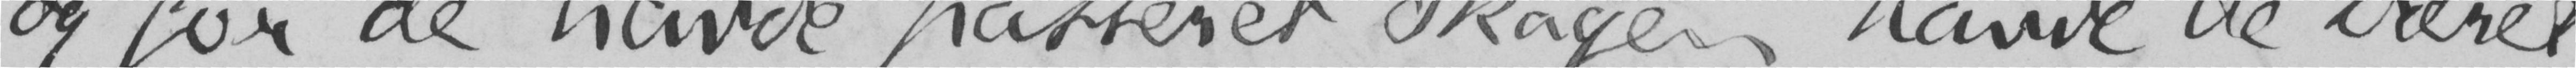og før de havde passeret Skagen, havde de været
